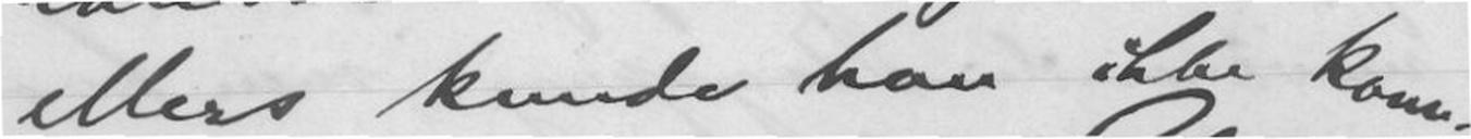ellers kunde han ikke kom-
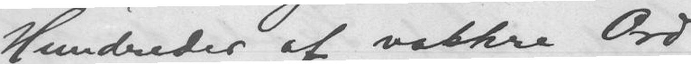Hundreder af vakkre Ord
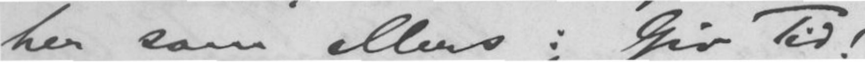her som ellers: Giv Tid!
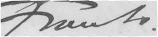Frants.
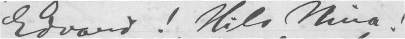Edvard! Hils Nina!
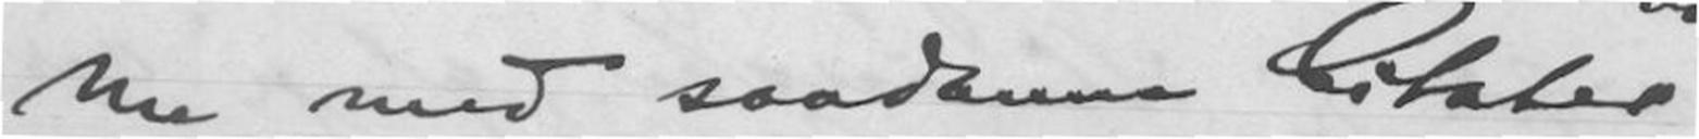me med saadanne Citater
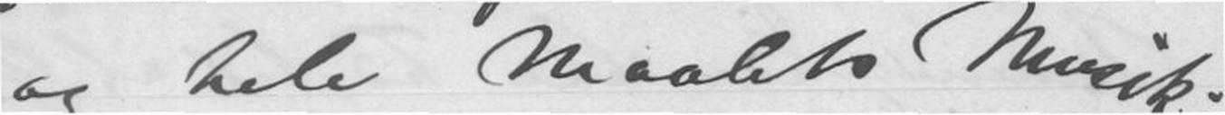og hele Maalets Musik.
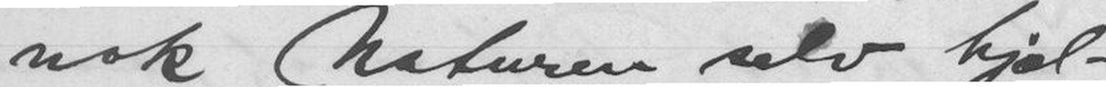nok Naturen selv hjæl-
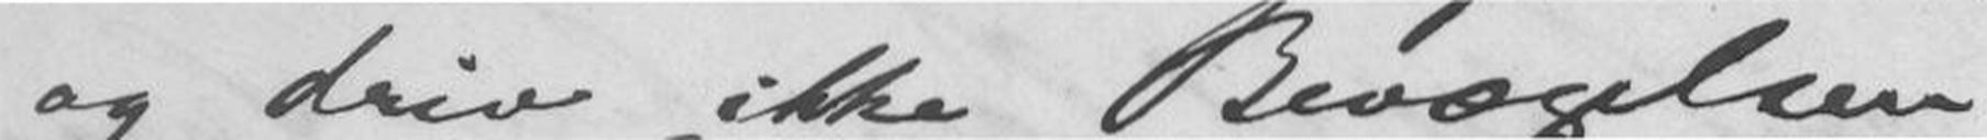og driv ikke Bevægelsen
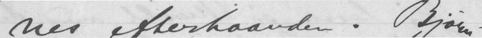nes efterhaanden. Bjørn-
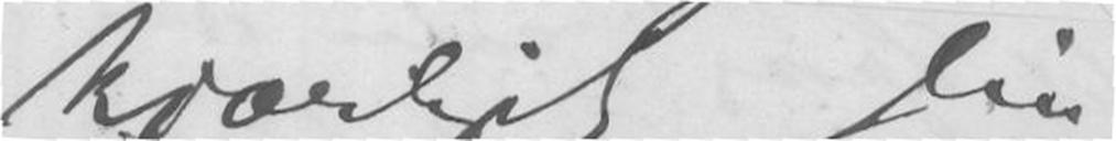kjærligst Din
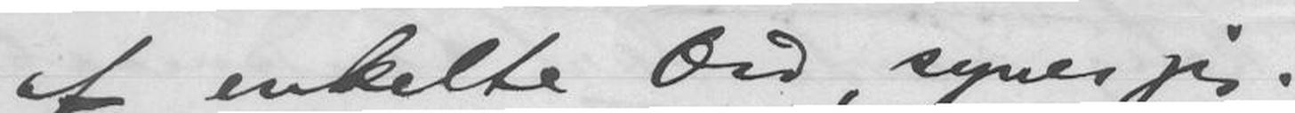af enkelte Ord, Synes jeg.
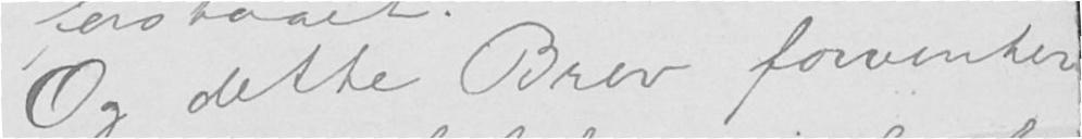Og dette Brev forventer
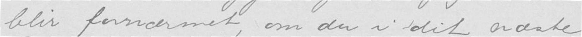blir fornærmet, om du i dit næste
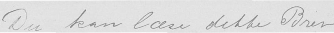Du kan læse dette Brev
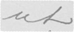ut
